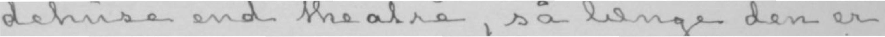dehuse end theatre, så længe den er
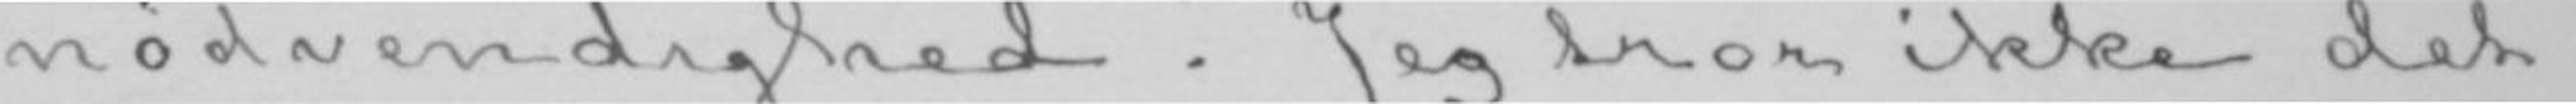nødvendighed. Jeg tror ikke det
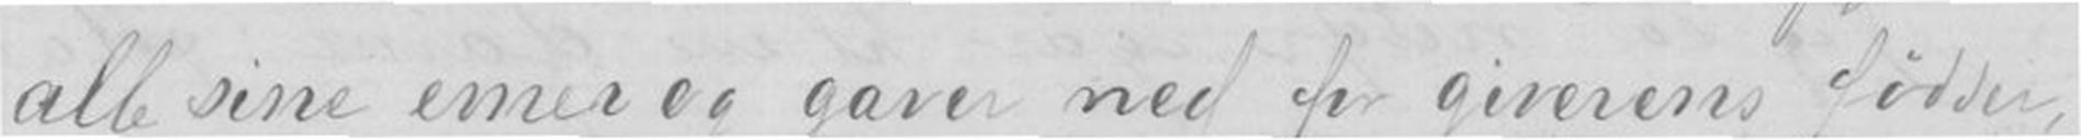alle sine evner og gaver ned for giverens fødder, -
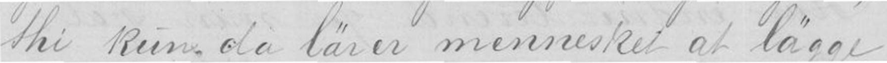thi kun da lärer mennesket at lägge
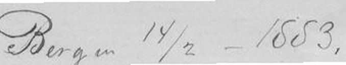Bergen 14/2 - 1883.
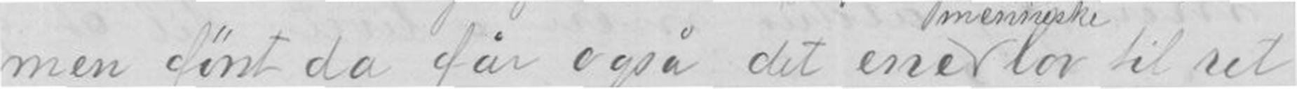men først da får også det ene lov til ret
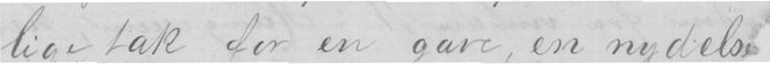lige tak for en gave, en nydels
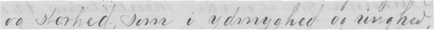og storhed, som i ydmyghed og ringhed;
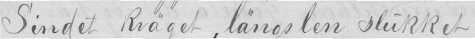Sindet kräget, längslen slukket
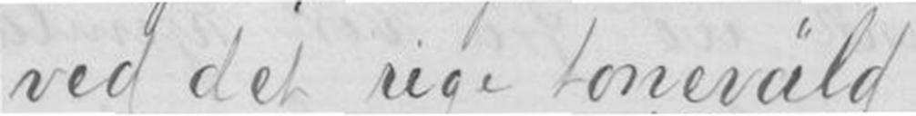ved det rige toneväld
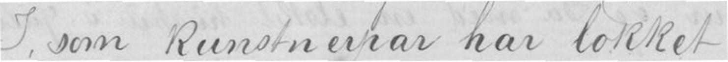I, som kunstnerpar har lokket
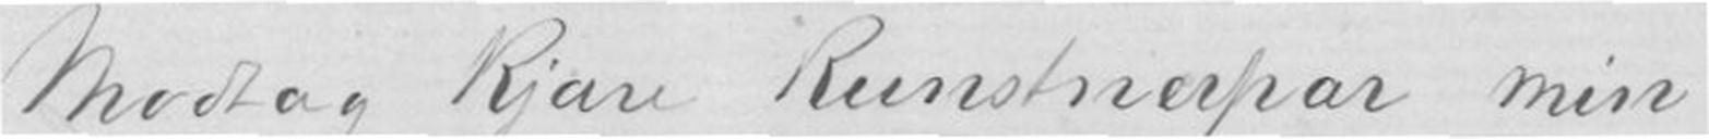Modtog kjære kunstnerpar min
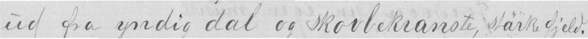ud fra yndig dal og skovbekranste, stärke fjeld.
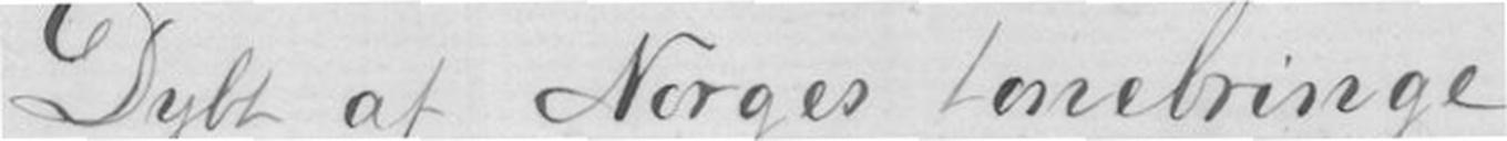Dybt af Norges tonebringe
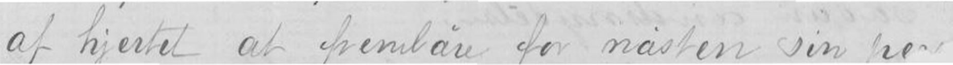af hjertet at frembäre for nästen sin peBit borte
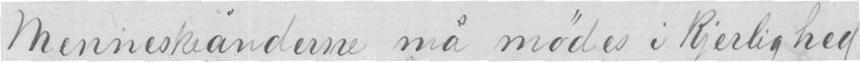Menneskeånderne må mødes i kjerlighed
